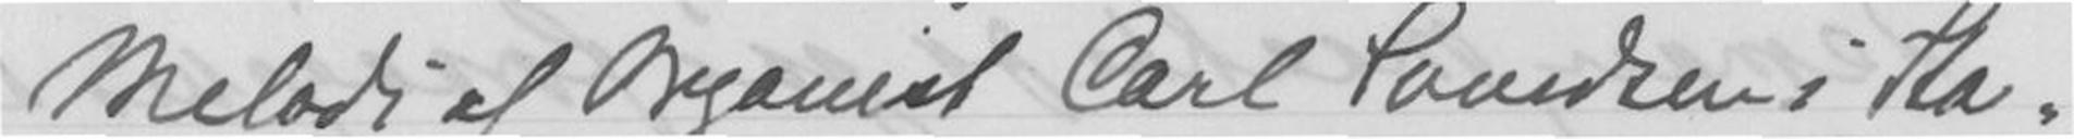Melodi af Organist Carl Svendsen i Sta-
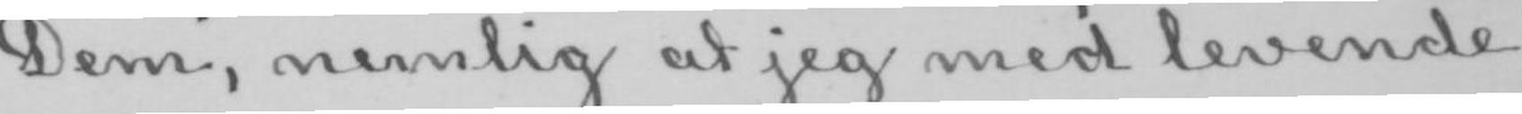Dem, nemlig at jeg med levende
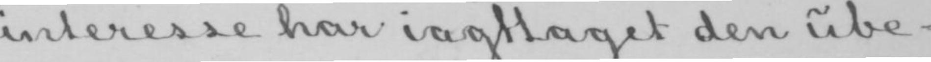interesse har iagttaget den ube-
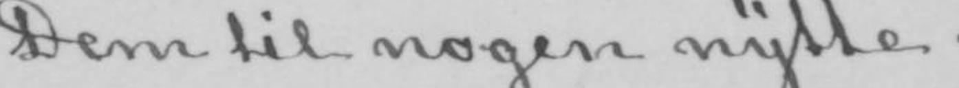Dem til nogen nytte.
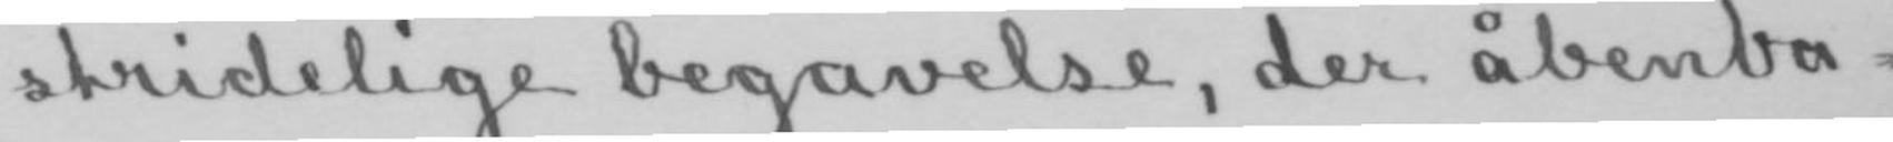stridelige begavelse, der åbenba-
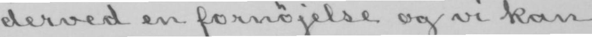derved en fornøjelse og vi kan
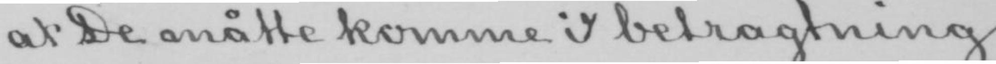at De måtte komme i betragtning
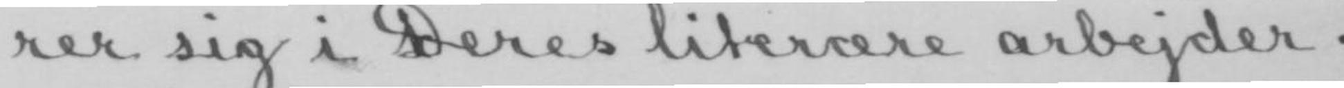rer sig i Deres literære arbejder.
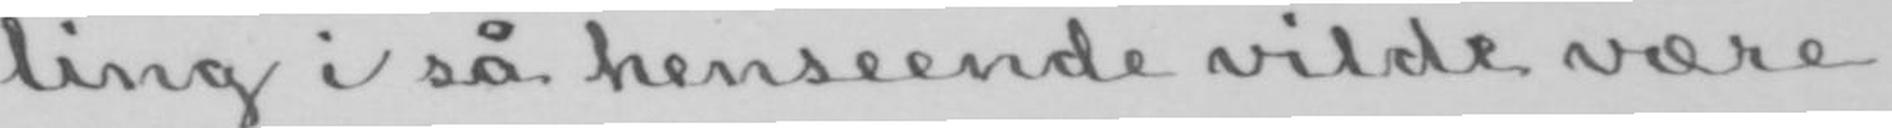ling i så henseende vilde være
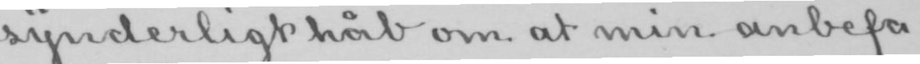synderligt håb om at min anbefa-
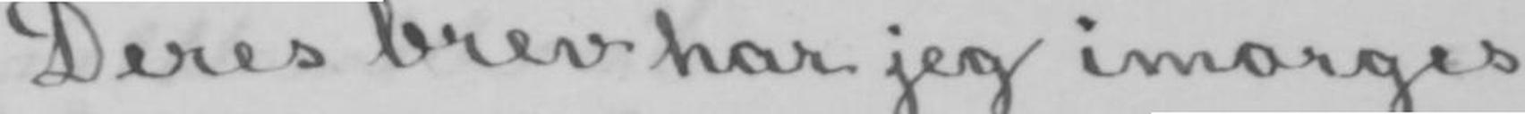Deres brev har jeg imorges
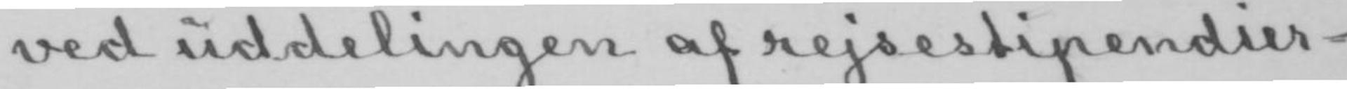ved uddelingen af rejsestipendier-
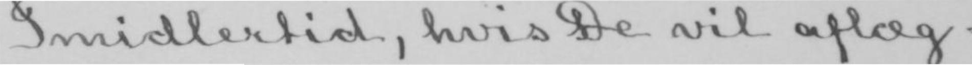Imidlertid, hvis De vil aflæg-
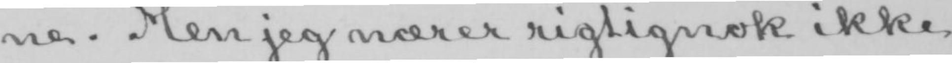ne. Men jeg nærer rigtignok ikke
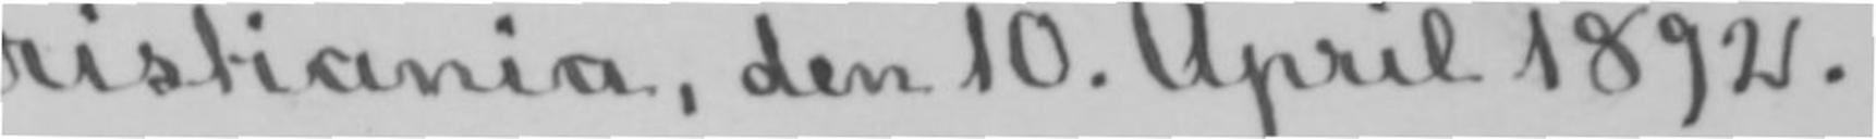Kristiania, den 10. April 1892.
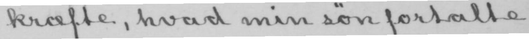kræfte, hvad min søn fortalte
